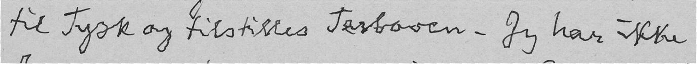til Tysk og tilstilles Terboven. Jeg har ikke
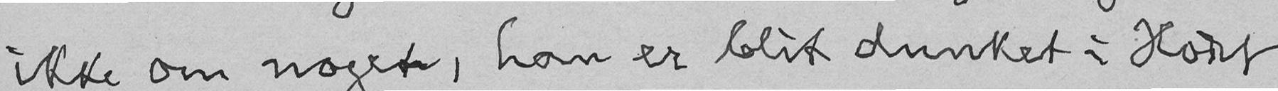ikke om noget, han er blit dunket i Hodet
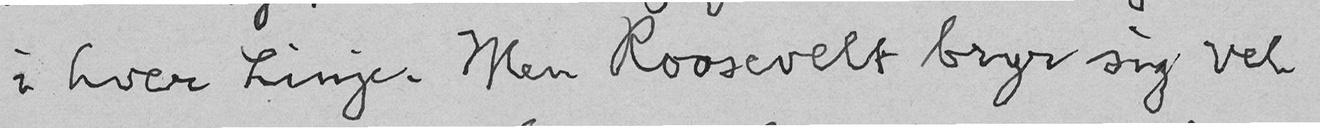i hver Linje. Men Roosevelt bryr sig vel
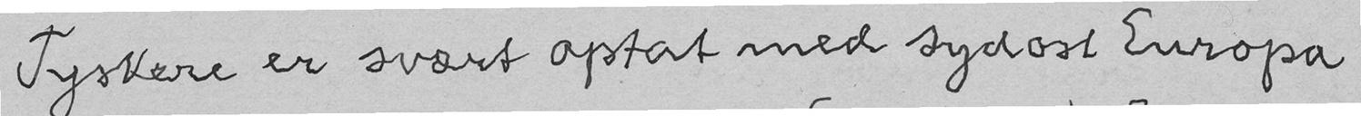Tyskere er svært optat med sydost Europa
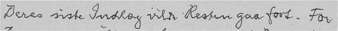Deres siste Indlæg vilde Resten gaa fort. For
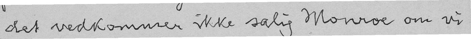det vedkommer ikke salig Monroe om vi
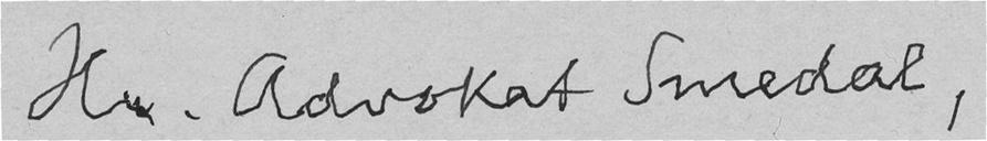Hr. Advokat Smedal,
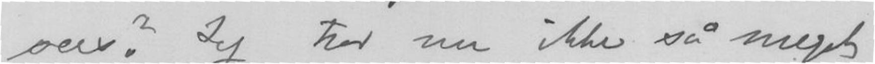sees? Jeg tror nu ikke så meget
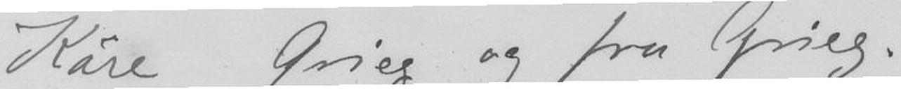Käre Grieg og fru Grieg.
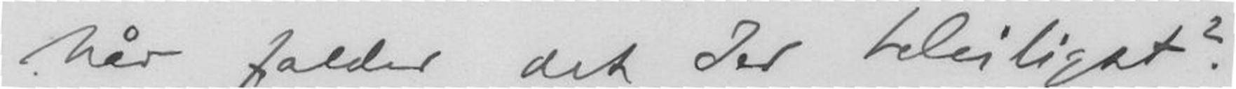Når falder det Jer beleiligst?
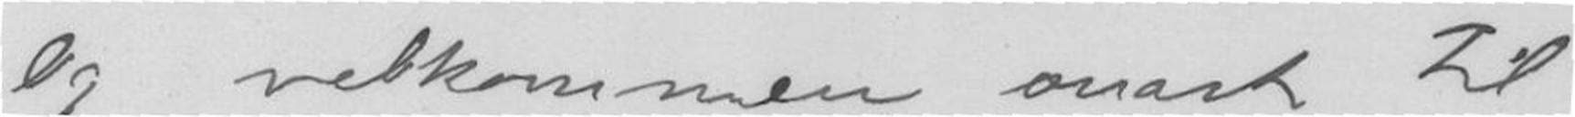Og velkommen snart til
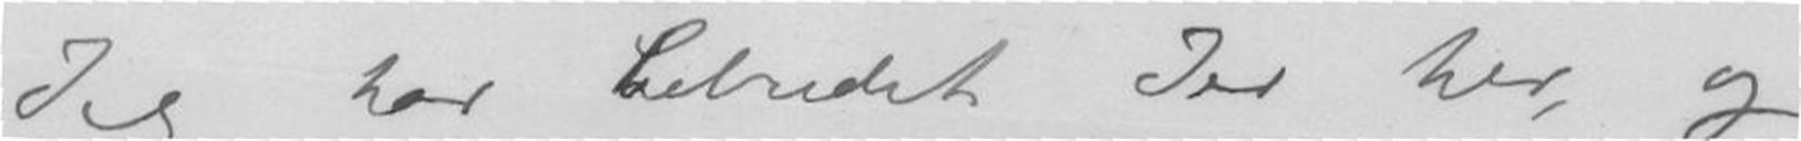Jeg har bebudet Jer her, og
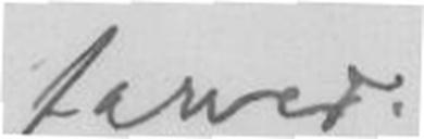farver.
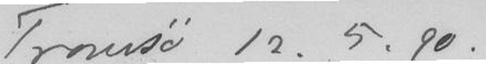Tromsø 12.5.90
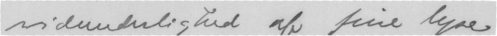vidunderlighed af fine lyse
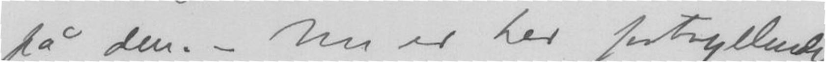på den. - Nu er her fortryllende
